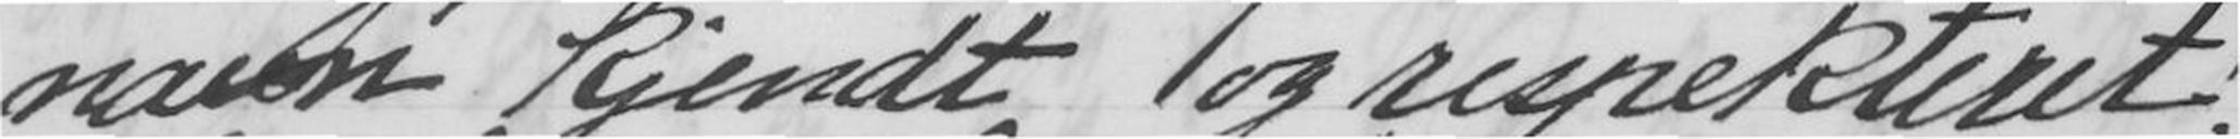navn kjendt og respekteret!
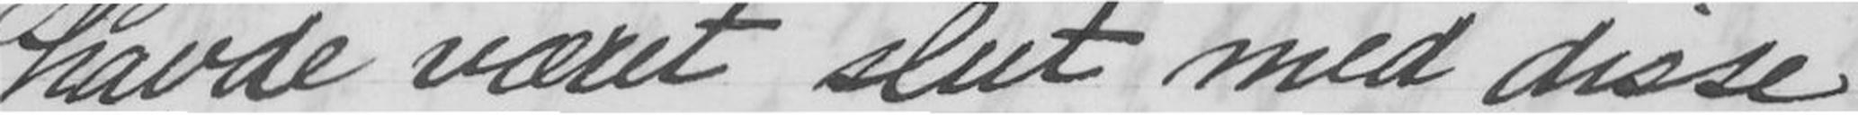havde været slut med disse
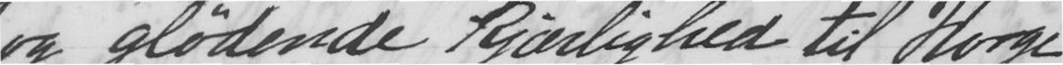og glødende kjærlighed til Norge
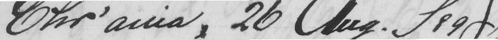Chr'ania, 26 Aug. 1898.
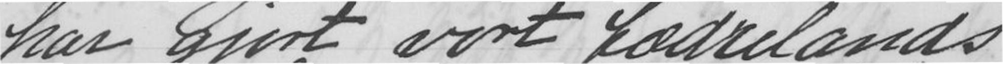har gjort vort fædrelands
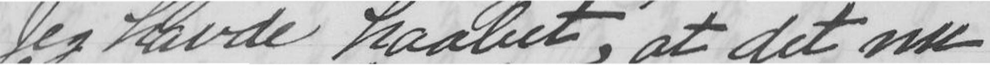Jeg havde haabet, at det nu
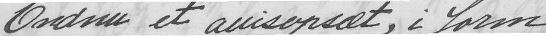Endnu et avisopsæt, i form
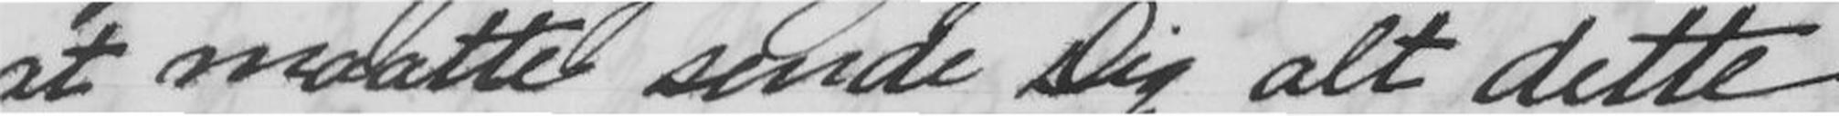at maatte sende Dig alt dette
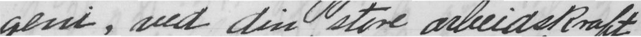geni ved din store arbeidskraft
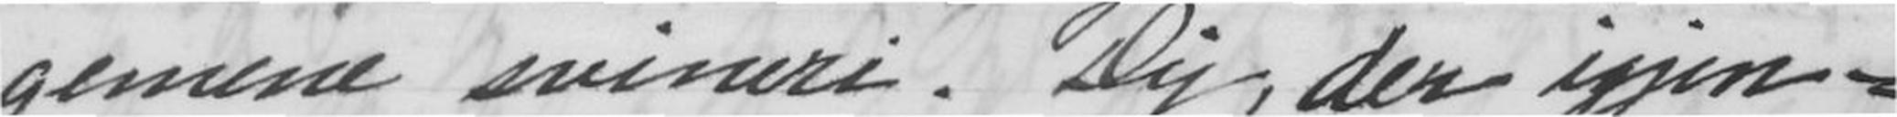gemene svineri. Dig, der igjen-
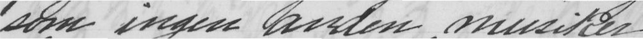som ingen anden musiker
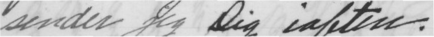sender jeg Dig iaften.
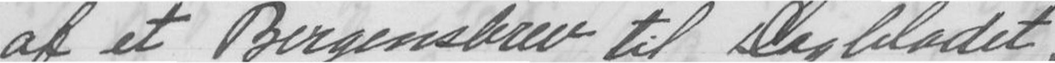af et Bergensbrev til Dagbladet,
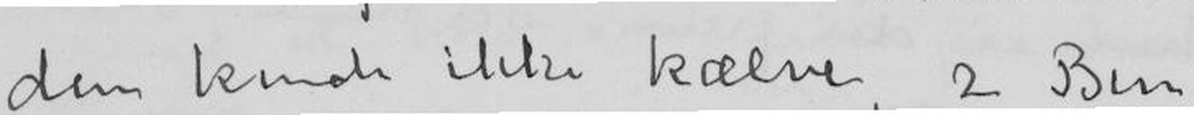den kunde ikke kælve, 2 Ben
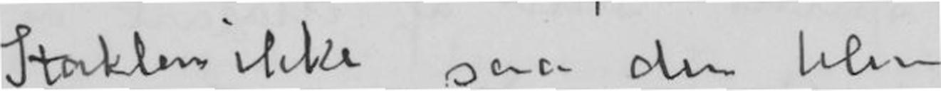Stoklen ikke, saa den blev
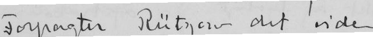Forpagter Rutgers det vide,
